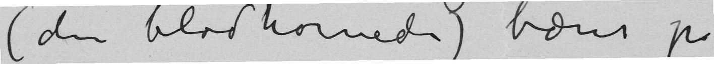(den blødhornede) bærer på
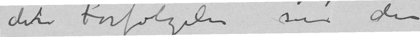den Forfølgelse som den
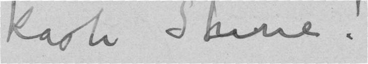kaste Stene!
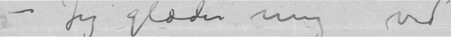– Jeg glæder mig ved
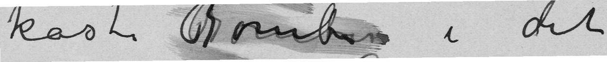kaste Bomber i det
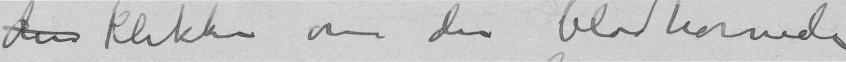den Klikken om den blødhornede
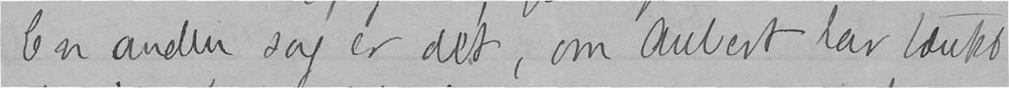En anden sag er det, om Aubert har tænkt
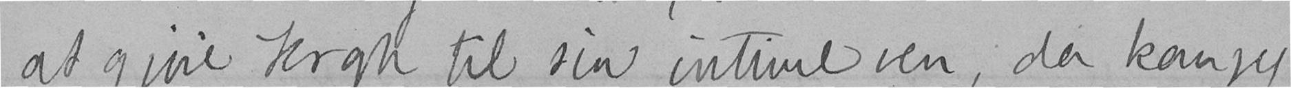at gjøre Krogh til sin intime ven, da kan jeg
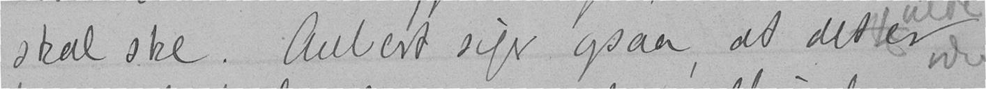skal ske. Aubert siger ogsaa at det er
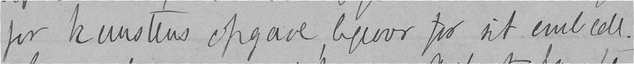for kunstens opgave, ligeover for sit embede.
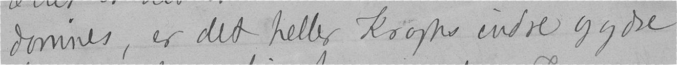dømmes, er det heller Kroghs indre og ydre
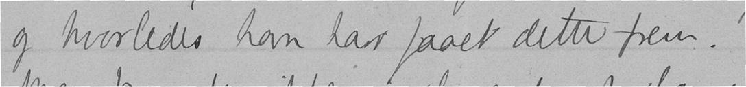og hvorledes han har faaet dette frem.
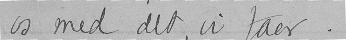os med det vi faar.
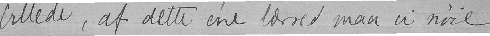billede, af dette ene lærred maa vi nøie
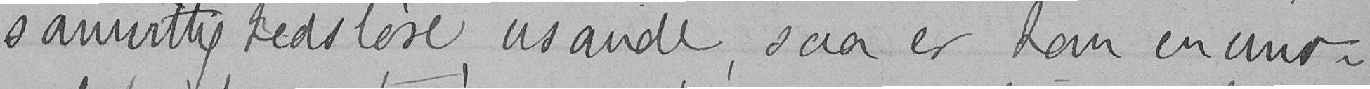samvittighedsløse, usande, saa er han en umo-
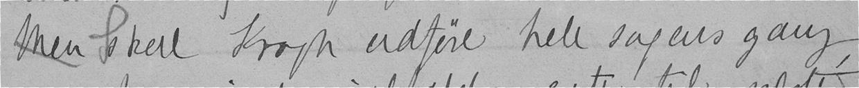Men skal Krogh udføre hele sagens gang,
